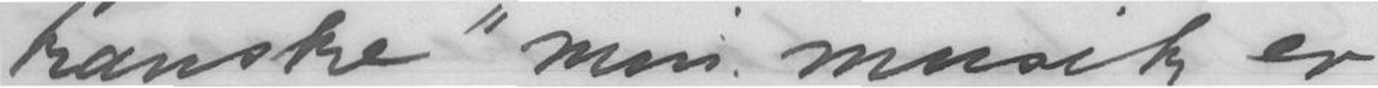kanske "min musik er
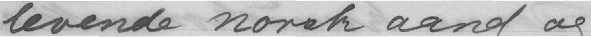levende norsk aand og
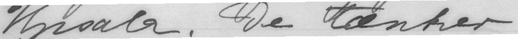Upsala. De tænker
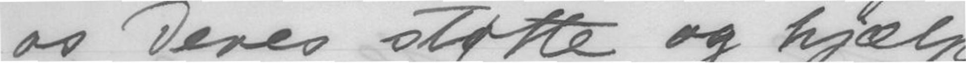os Deres støtte og hjælp
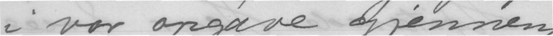i vor opgave gjennem
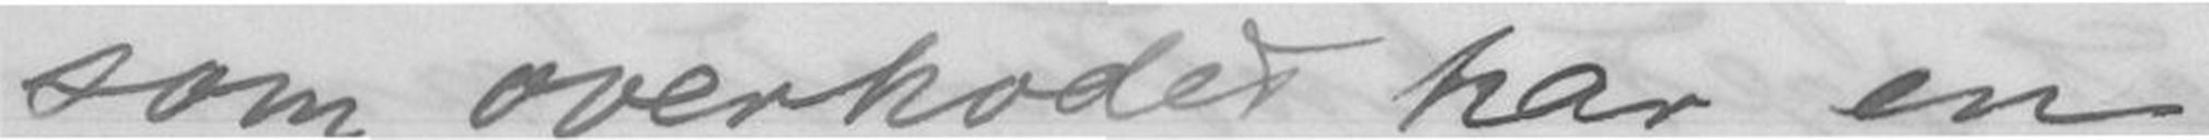som overhodet har en
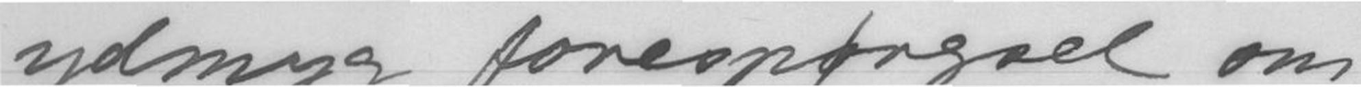ydmyg forespørgsel om
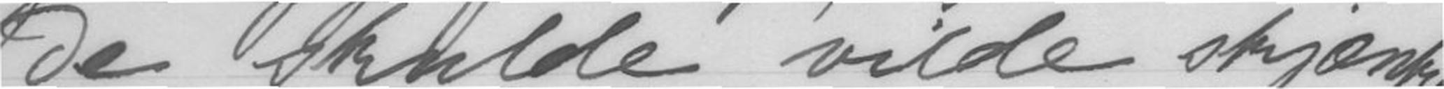De skulde vilde skjænke
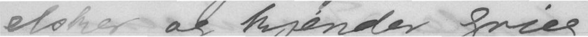elsker og kjender Grieg
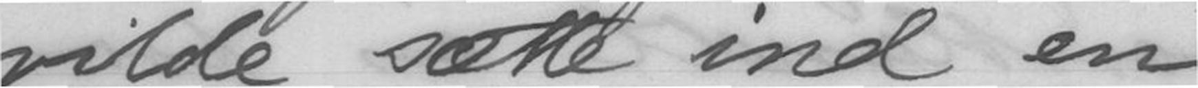vilde sætte ind en
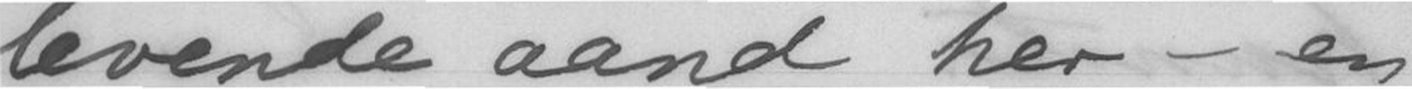levende aand her - en
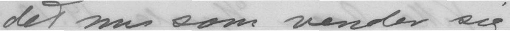det nu som vender sig
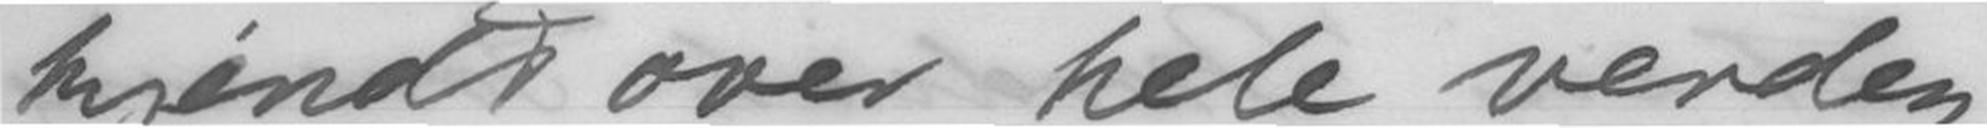kjendt over hele verden
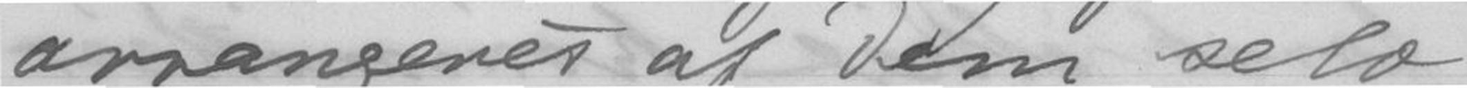arrangeret af Dem selv
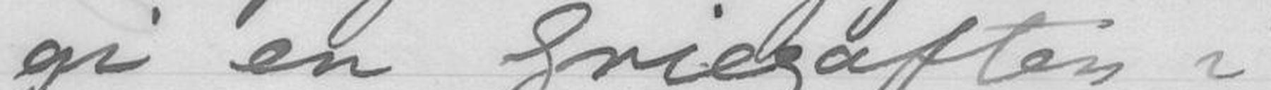gi en Griegaften i
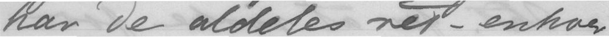har De aldeles ret - enhver
